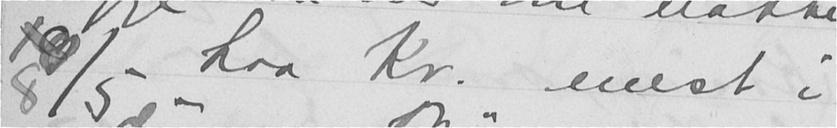8/5 Laa Kr. mest i
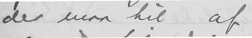der maa til af
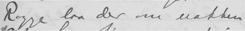Rogge laa der om natten.
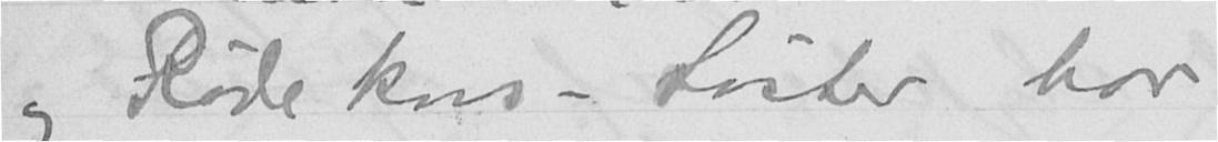og Røde kors-Søster har
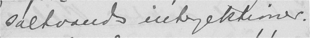saltvands indjektioner.
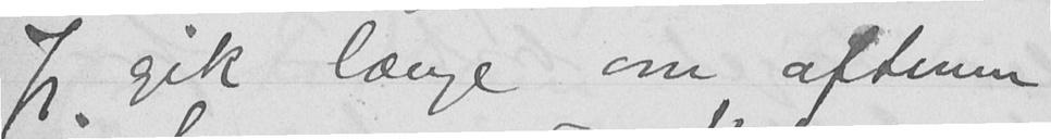Jeg gik længe om aftenen
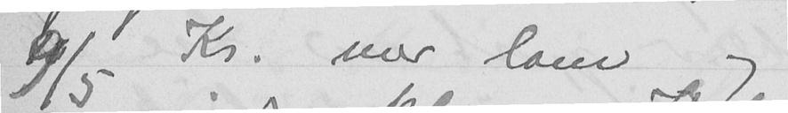9/5 Kr. var lam og
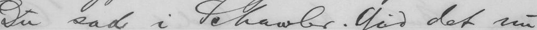Du sad i Schawler. Gid det nu
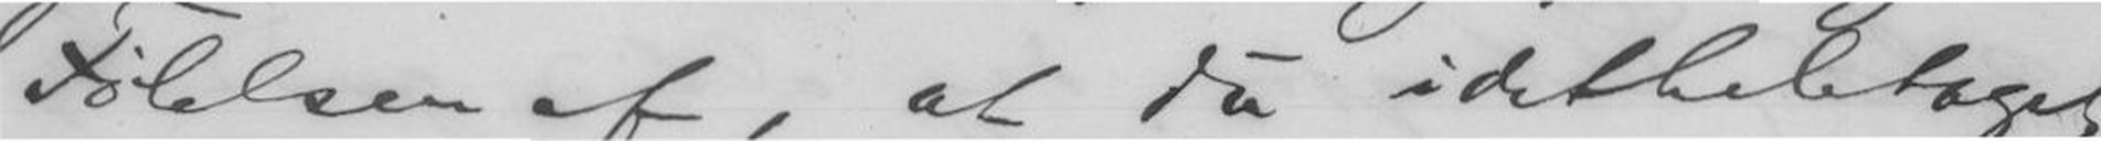Følelsen af, at du idetheletaget
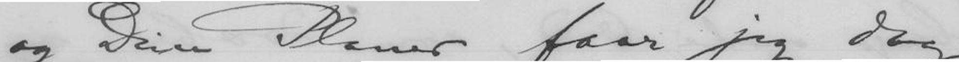og Dine Planer faar jeg dog
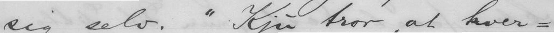sig selv. "Kju tror, at hver-
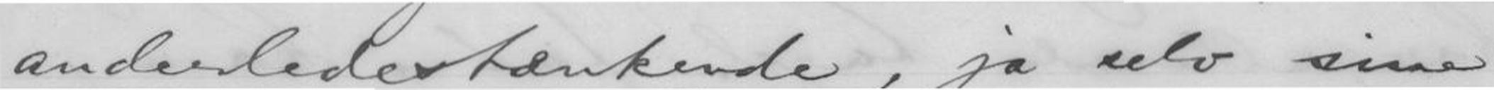anderledestænkende, ja selv sine
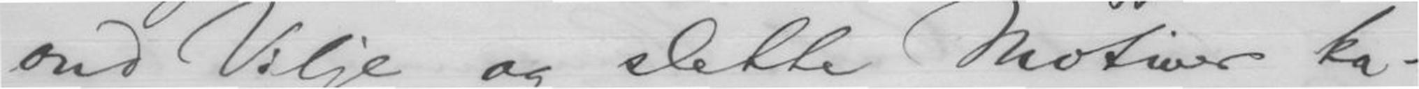ond Vilje og slette Motiver ka-
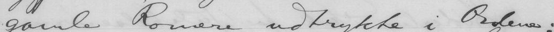gamle Romere udtrykte i Ordene:
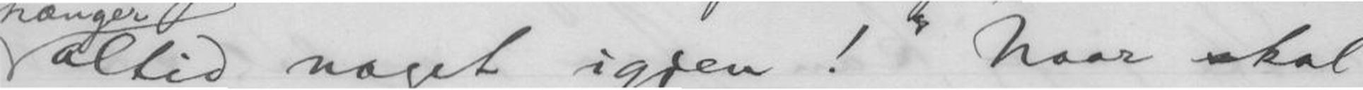altid noget igjen!" Naar skal
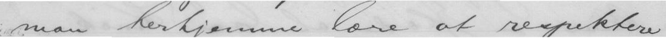man herhjemme lære at respektere
